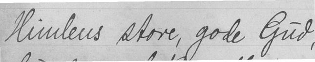Himlens store, gode Gud,
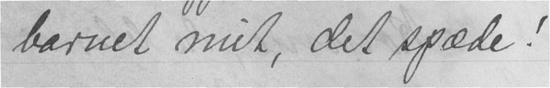barnet mit, det spæde!
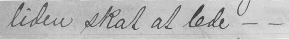liden skat at lede - -
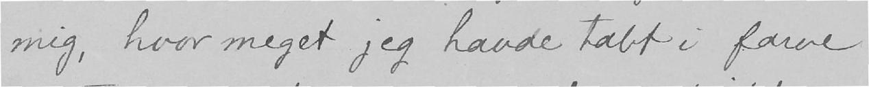mig, hvor meget jeg havde tabt i farve
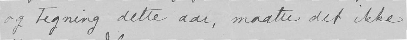og tegning dette aar, maatte det ikke
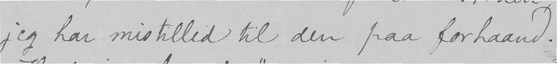jeg har mistillid til den paa forhaand.
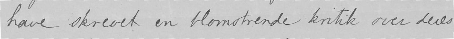have skrevet en blomstrende kritik over deres
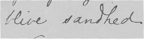blive sandhed.
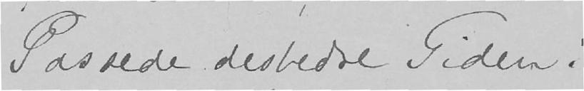Passede desbedre Tiden:
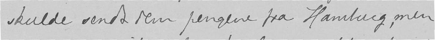skulde sendt dem pengene fra Hamburg, men
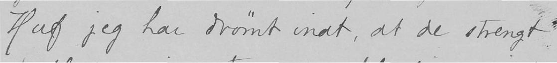Huf jeg har drømt inat, at de strengt
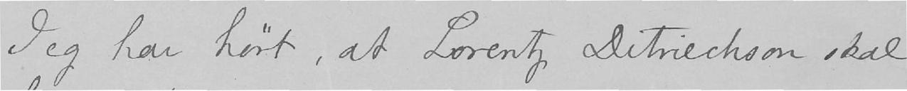Jeg har hørt, at Lorentz Ditriechson skal
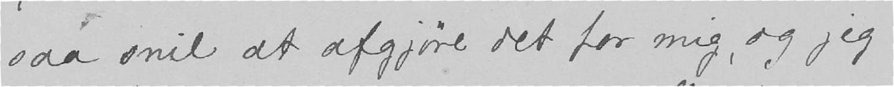saa snil at afgjøre det for mig, og jeg
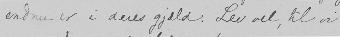endnu er i deres gjæld. Lev vel, til vi
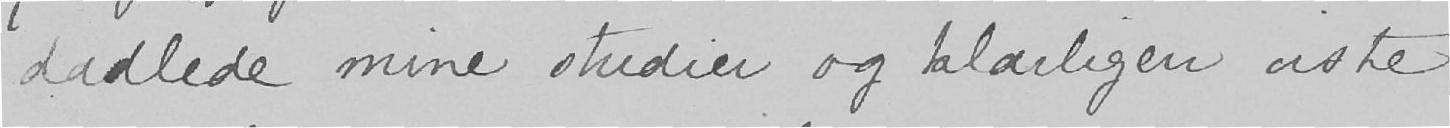dadlede mine studier og klarligen viste
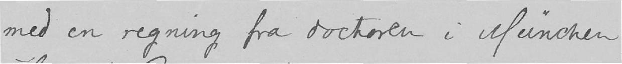med en regning fra doctoren i München,
